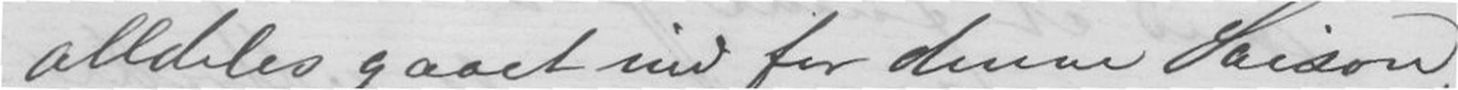alldeles gaaet ind for denne Saison,
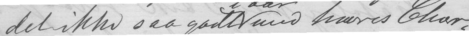det ikke saa godt med hvores Chor-
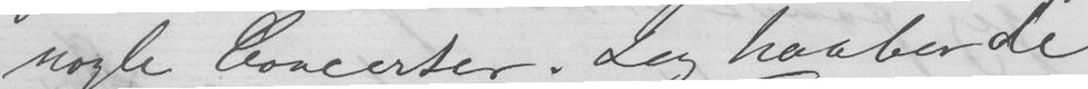nogle Concerter. Jeg haaber de
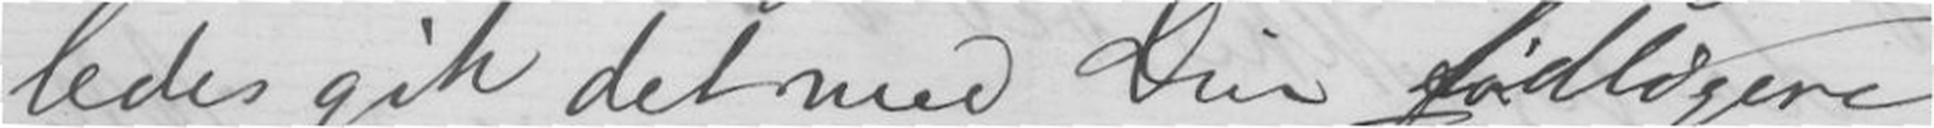ledes gik det med Din Tidligere
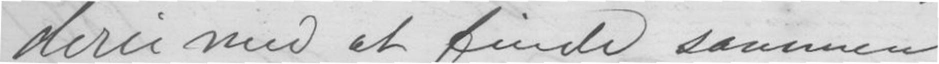derie med at finde sammen
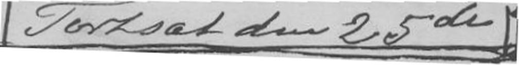|Fortsat den 25de
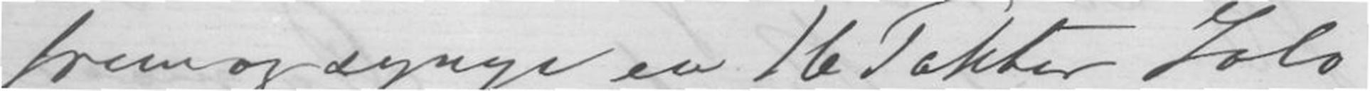frem og synge en 16 Takter Solo
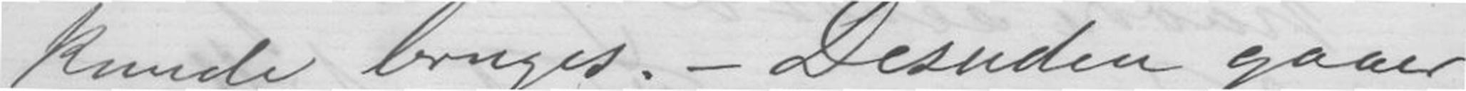kunde bruges. - Desuden gaaer
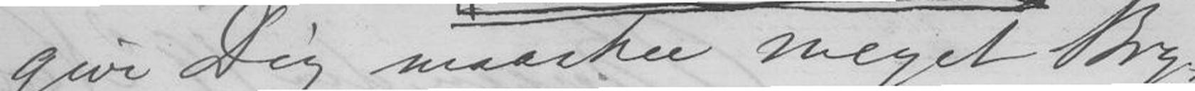give Dig maaskee meget Bry-
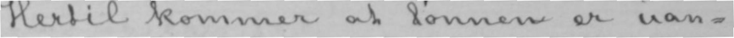Hertil kommer at lønnen er uan-
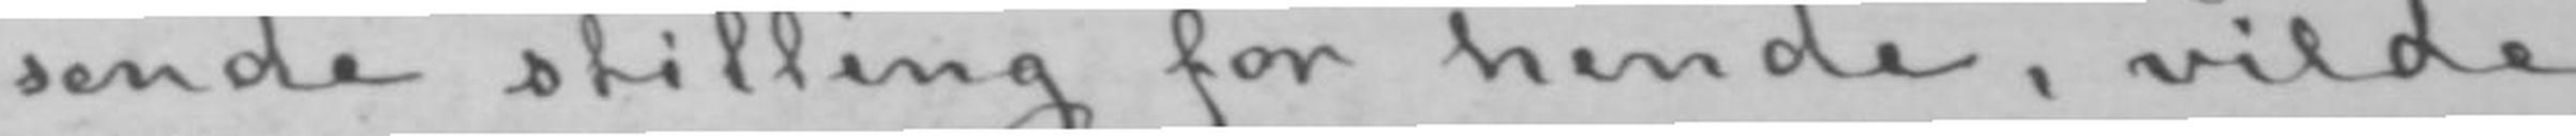sende stilling for hende, vilde
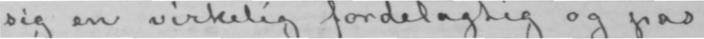sig en virkelig fordelagtig og pas-
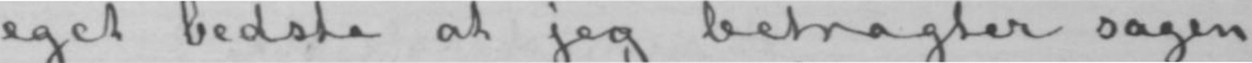eget bedste at jeg betragter sagen
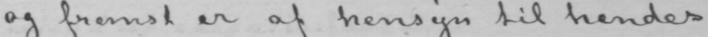og fremst er af hensyn til hendes
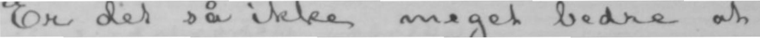Er det så ikke meget bedre at
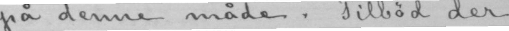på denne måde. Tilbød der
